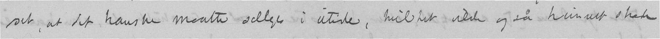set, at det kanske maatte sælges i utide, hvilket vilde også kunnet skade
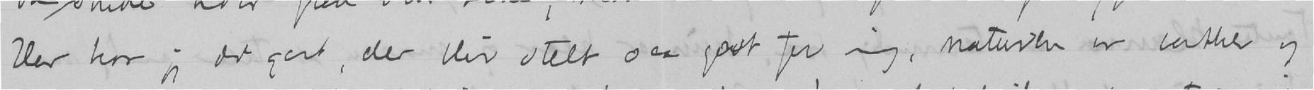Her har jeg det godt, der blir stelt saa godt for mig, naturen er vakker og
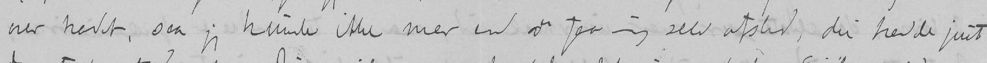over hodet, saa jeg kunde ikke mer end at faa mig selv afsted, du havde just
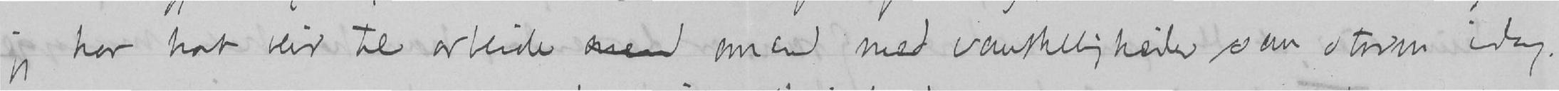jeg har hat veir til arbeide omed omend med vanskeligheder som storm idag.
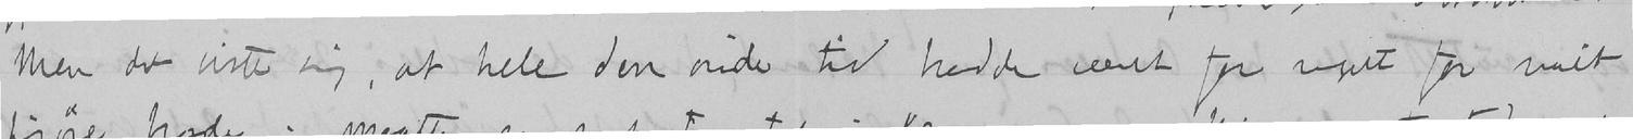Med det viste sig, at hele den onde tid havde været for meget for mit
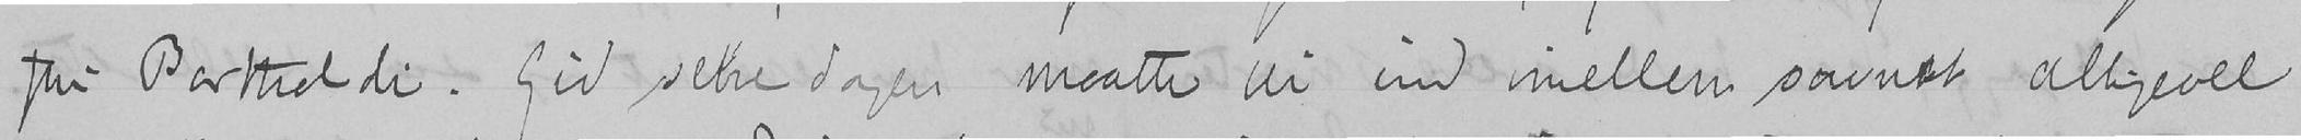fru Bartholdi. Gid selve dagen maatte bli ind imellem savnet alligevel
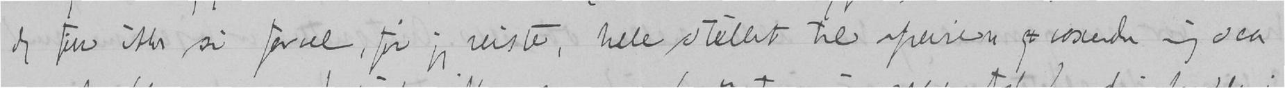Jeg fik ikke si farvel, før jeg reiste, hele stellet til afreisen g voxede mig saa
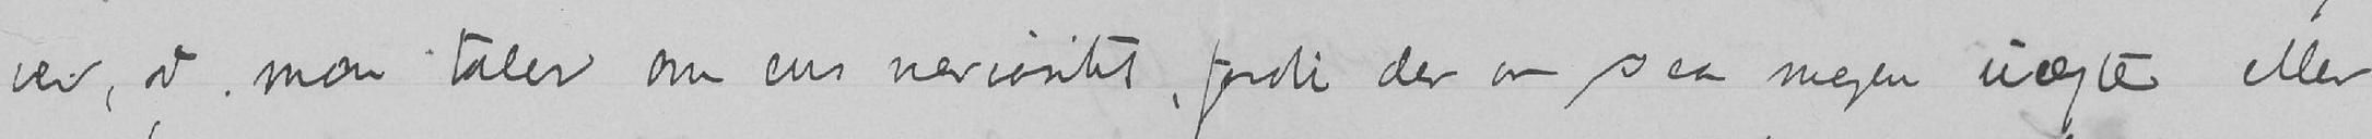ved, at man taler om ens nervøsitet, fordi der er saa megen uægte eller
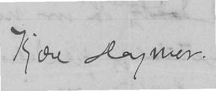Kjære Dagmar.
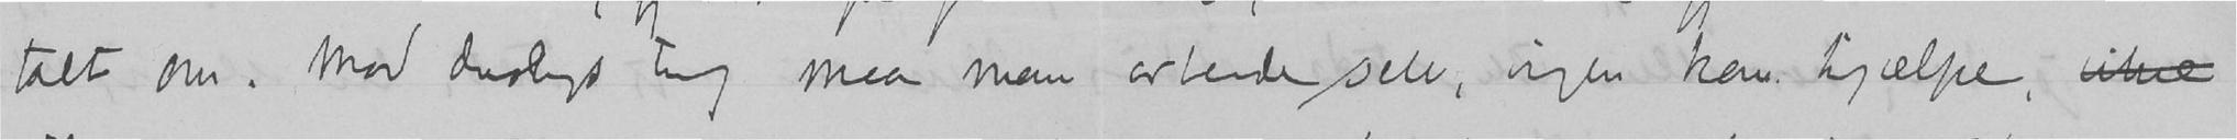talt om. Mod denslags ting maa man arbeide selv, ingen kan hjælpe, vilne
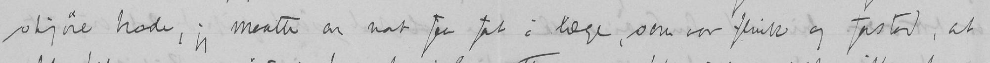skjøre hode, jeg maatte en nat faa tak i læge, som var flink og forstod, at
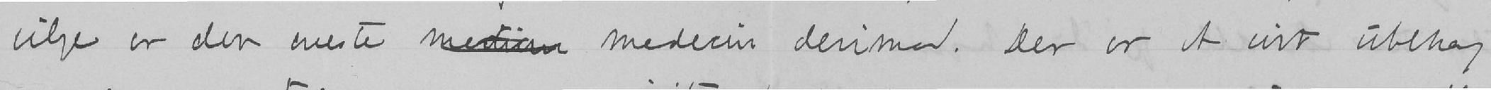vilje er den eneste medicin medecin derimod. Der er et vist ubehag
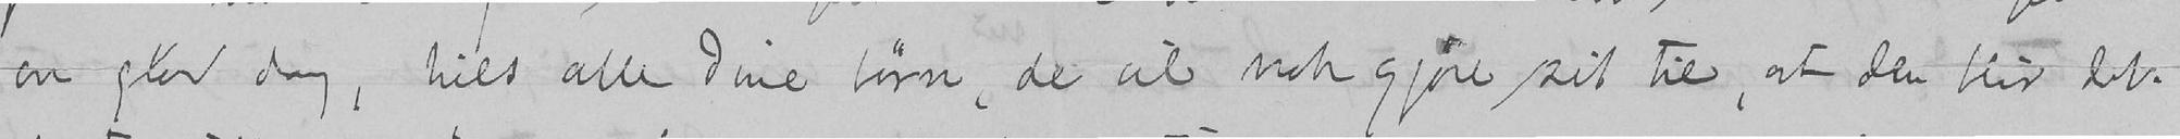en glad dag, hils alle Dine børn, de vil nok gjøre sit til, at den blir det.
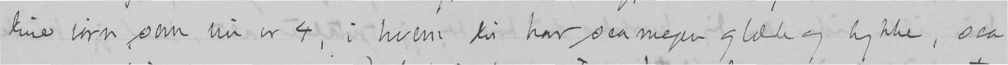dine børn som nu er 4, i hvem du har saamegen glæde og lykke, saa
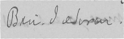Bru. Jæderen.
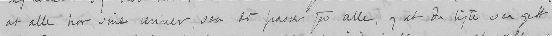at alle har sine venner, saa det passer for alle, og at du ligte saa godt
